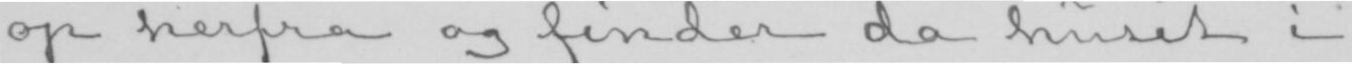op herfra og finder da huset i
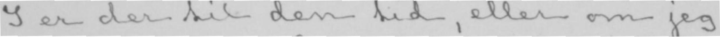I er der til den tid, eller om jeg
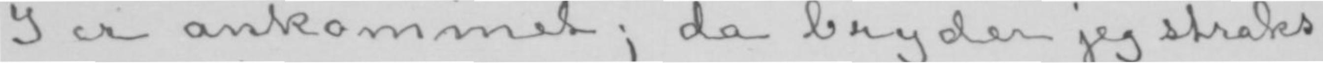I er ankommet; da bryder jeg straks
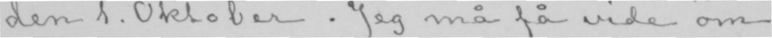den 1. Oktober. Jeg må få vide om
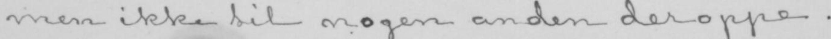men ikke til nogen anden deroppe.
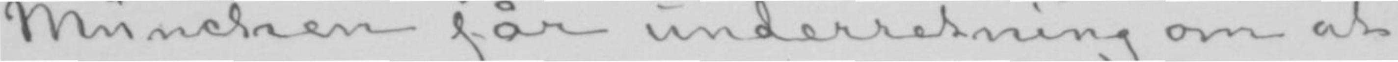München får underretning om at
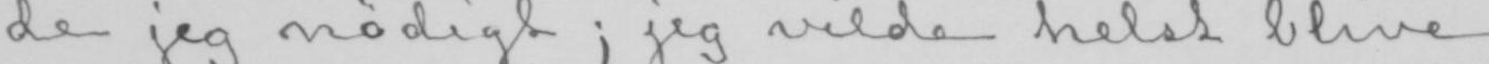de jeg nødigt; jeg vilde helst blive
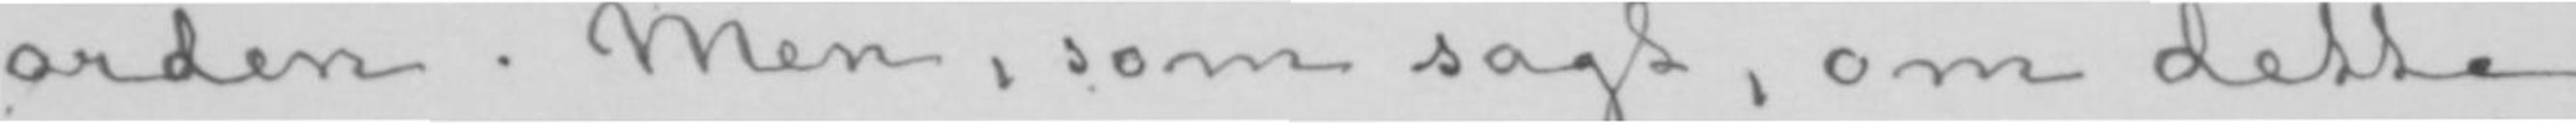orden. Men, som sagt, om dette
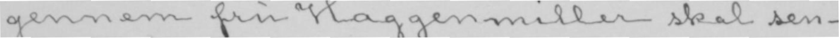gennem fru Haggenmiller skal sen-
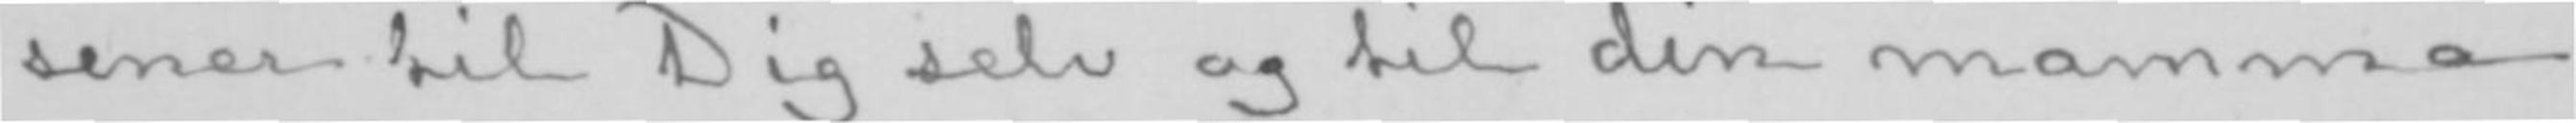sener til Dig selv og til din mamma,
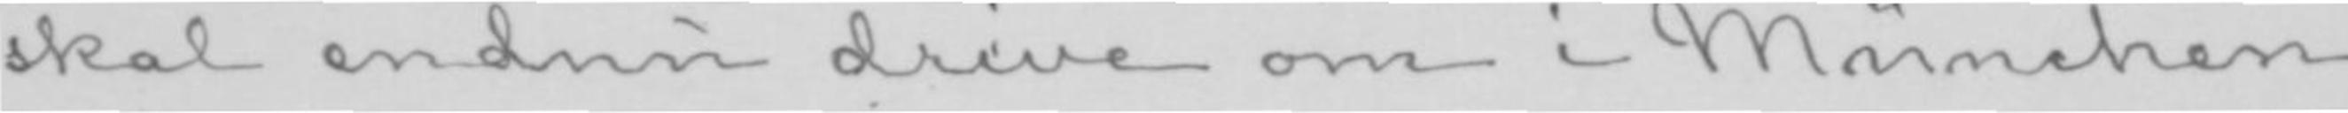skal endnu drive om i München
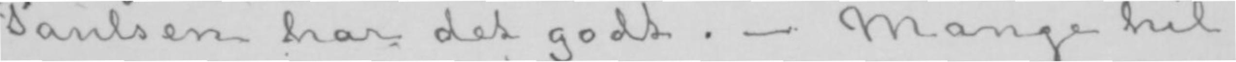Paulsen har det godt.- Mange hil-
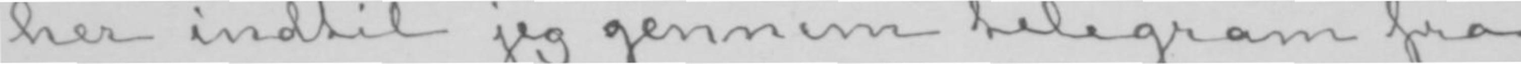her indtil jeg gennem telegram fra
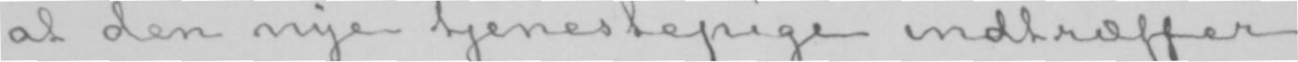at den nye tjenestepige indtræffer
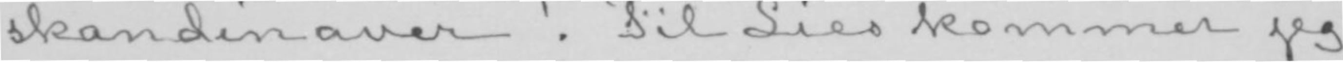skandinaver! Til Lies kommer jeg
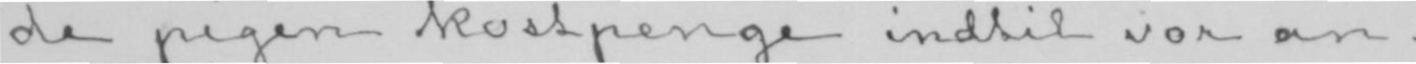de pigen kostpenge indtil vor an-
